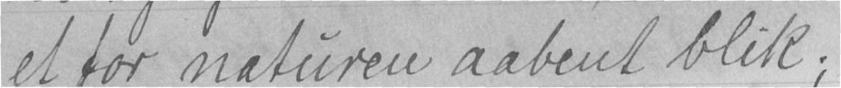et for naturen aabent blik;
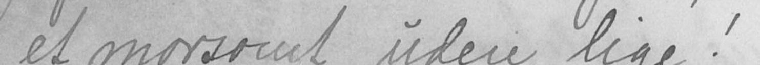et morsomt, uden lige!
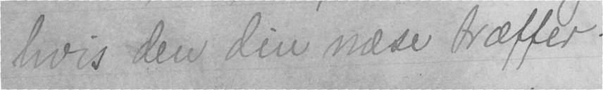hvis den din næse træffer!
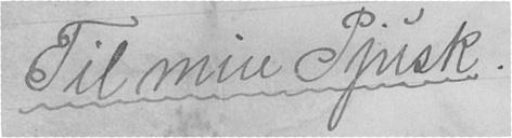Til min Pjusk.
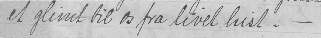et glimt til os fra livet hist. -
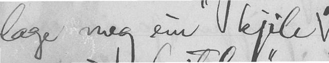lage mig en kjæle
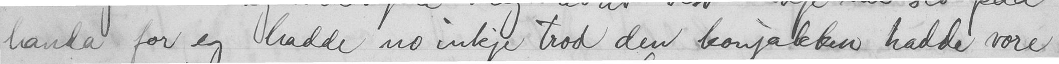handa for eg hadde no inkje trod den konjakken hadde vore
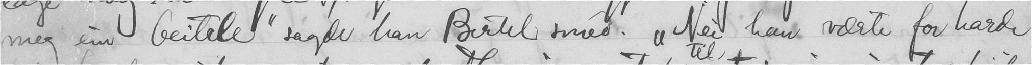meg ein beitele" sagde han Bertel smed. "Nei han værte for harde
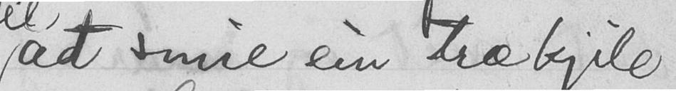at smie ein trækjile
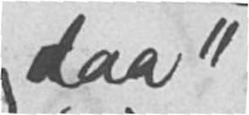daa"
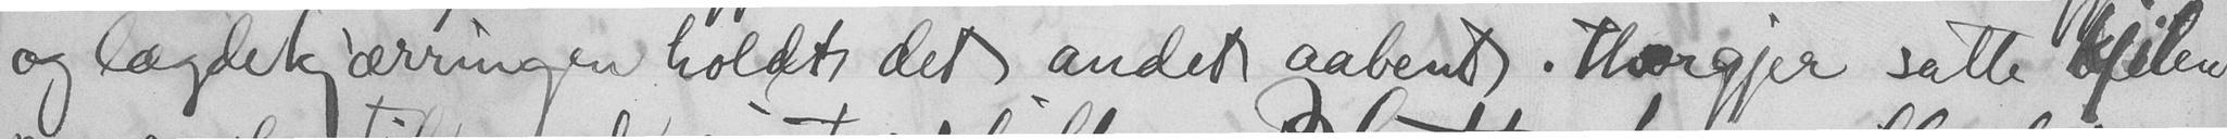og lægdekjærringen holdt det andet aabent. Thorgjer satte kjilen
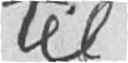til
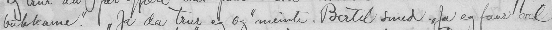bukkarne". "Ja da trur eg og" meinte Bertil smed. "Ja eg faar vel
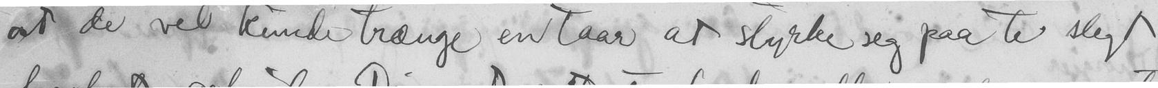at de vel kunde trænge en taar at styrke seg paa te sligt
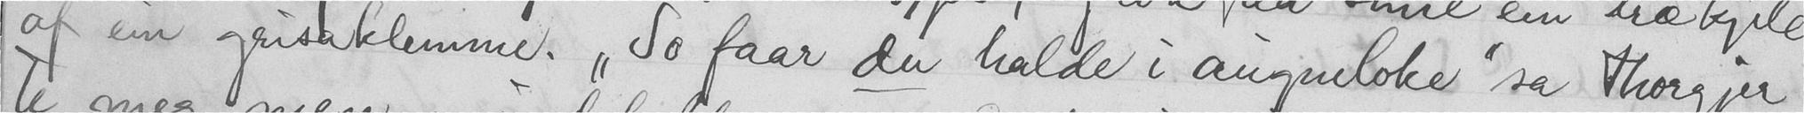at ein griseklemme. "So faar du halde i augneloke" sa Thorgjer
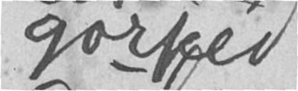gorped
